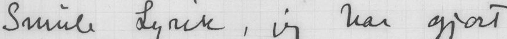Smule Lyrik, jeg har gjort
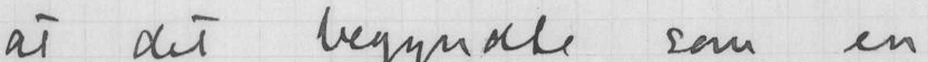at det begyndte som en
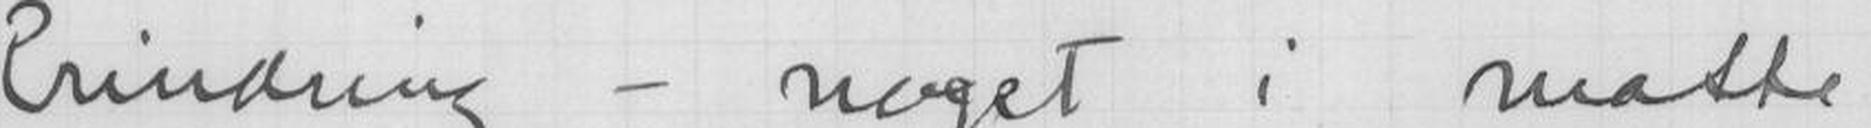Erindring - noget i matte
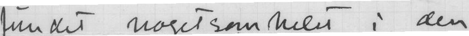fundet nogetsomhelst i den
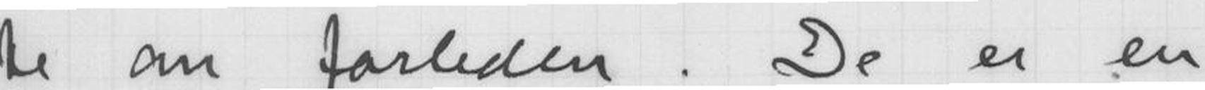te om forleden. De er en
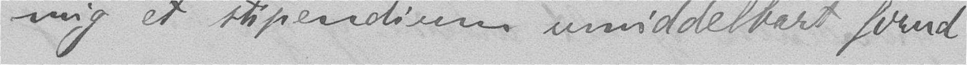mig et stipendium umiddelbart forud
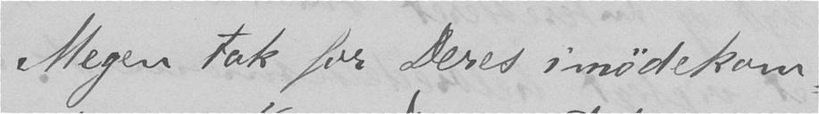Megen tak for Deres imødekom-
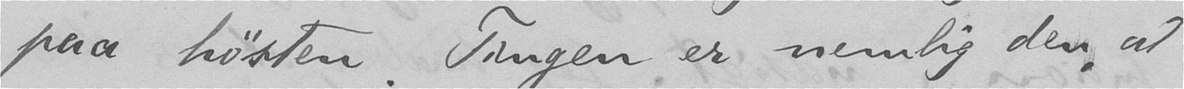paa høsten. Tingen er nemlig den, at
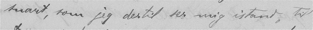snart, som jeg dertil ser mig istand, til
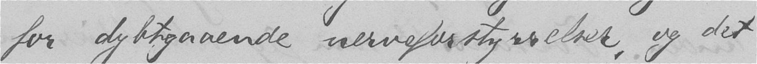for dybtgaaende nerveforstyrrelser, og det
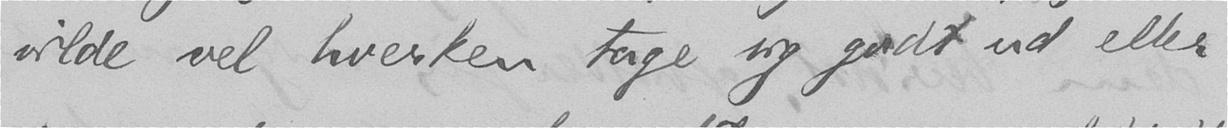vilde vel hverken tage sig godt ud eller
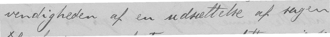vendigheden af en udsættelse af sagen
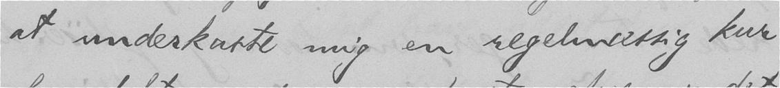at underkaste mig en regelmæssig kur
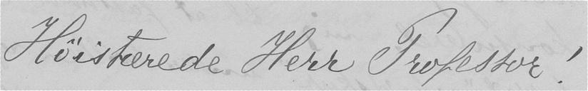Høistærede Herr Professor!
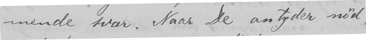mende svar. Naar De antyder nød-
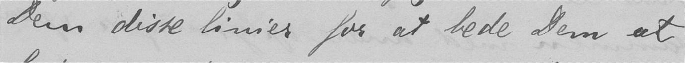Dem disse linier for at bede Dem at
